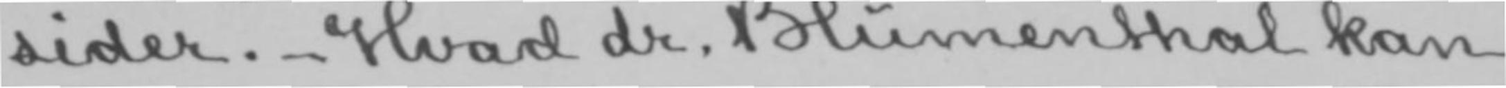sider.- Hvad dr. Blumenthal kan
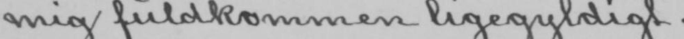mig fuldkommen ligegyldigt.
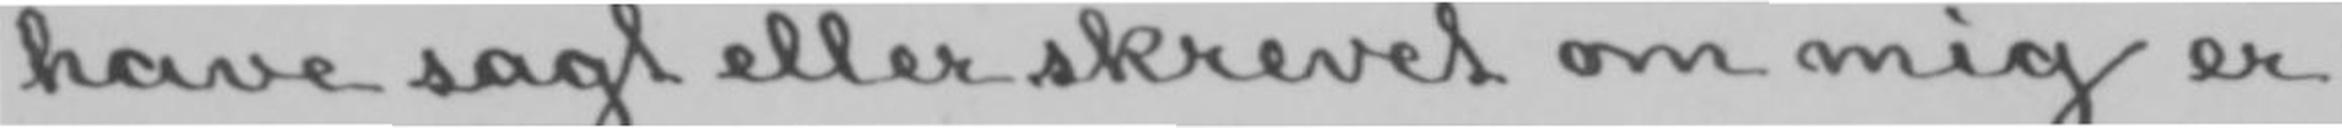have sagt eller skrevet om mig er
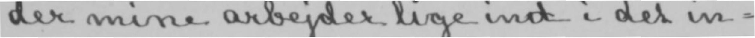der mine arbejder lige ind i det in-
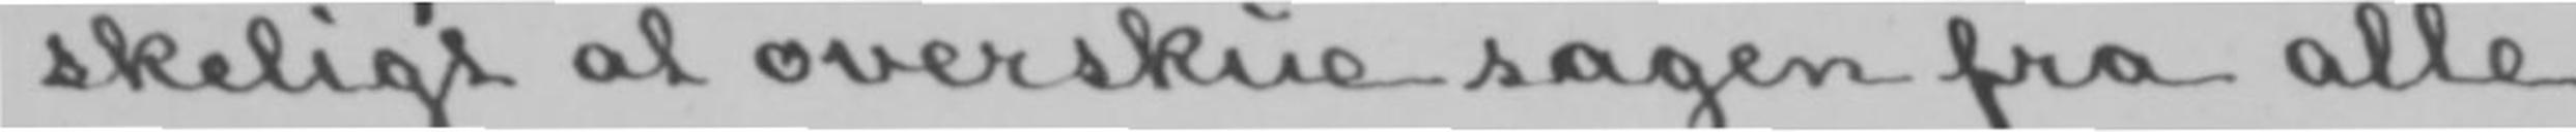skeligt at overskue sagen fra alle
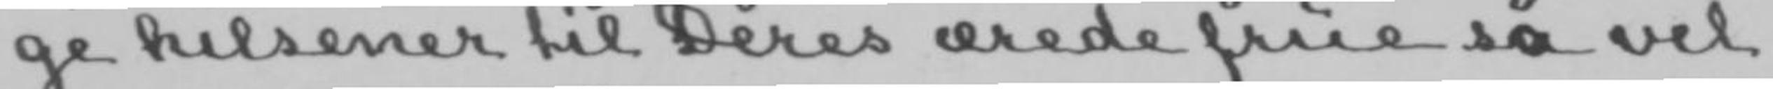ge hilsener til Deres ærede frue så vel
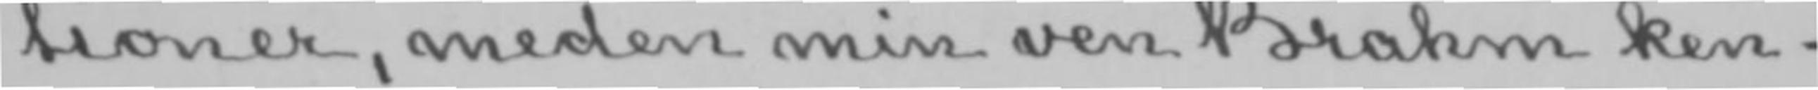tioner, meden min ven Brahm ken-
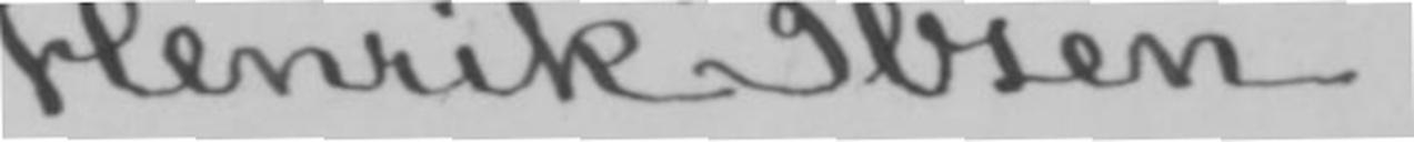Henrik Ibsen.
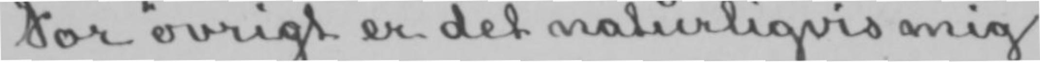For øvrigt er det naturligvis mig
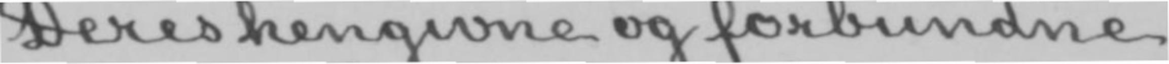Deres hengivne og forbundne
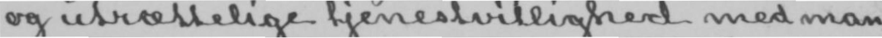og utrættelige tjenestvillighed med man-
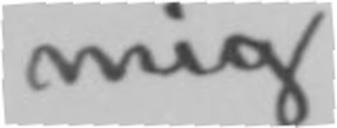mig
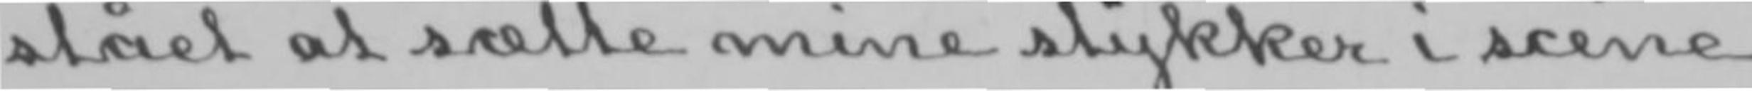stået at sætte mine stykker i scene
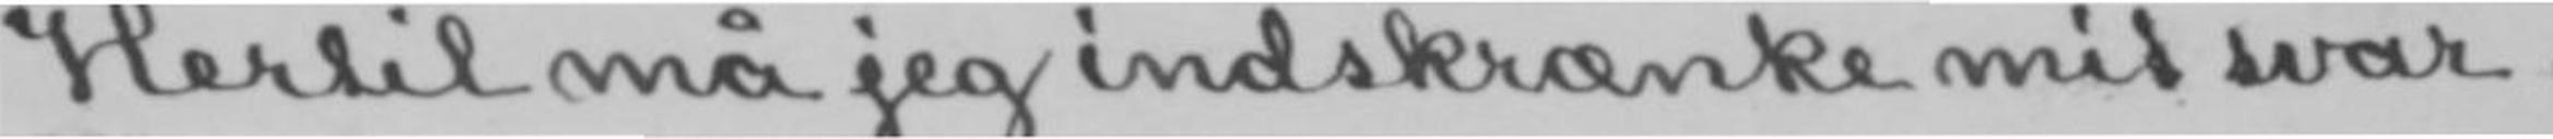Hertil må jeg indskrænke mit svar.
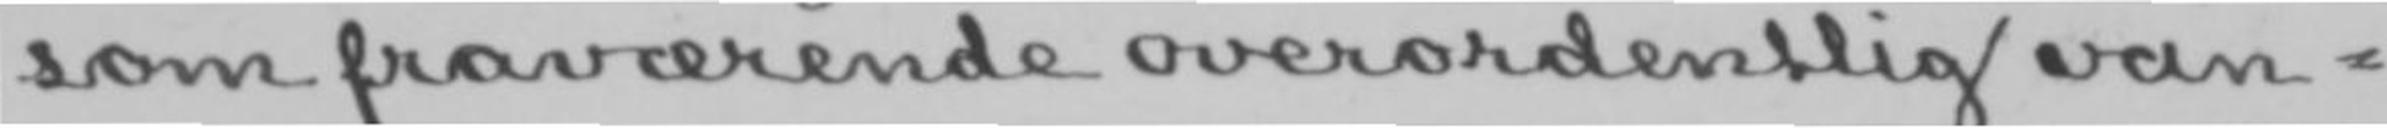som fraværende overordentlig van-
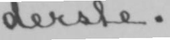derste.
